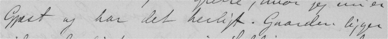Gjæst og har det herligt. Gaarden ligger
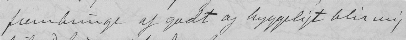frembringe af godt og hyggeligt blir mig
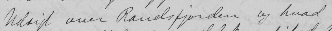Udsigt over Randsfjorden og hvad
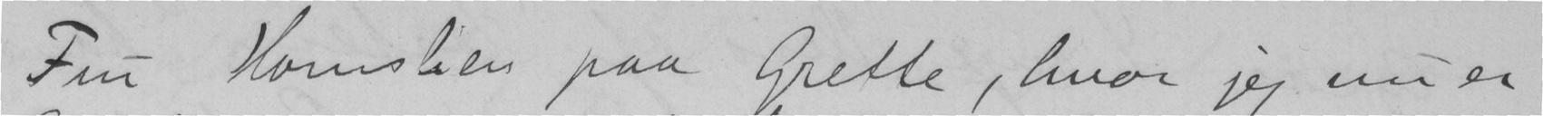Fru Homslien paa Grette, hvor jeg nu er
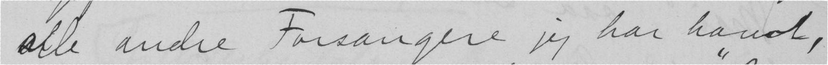alle andre Forsangere jeg har havt,
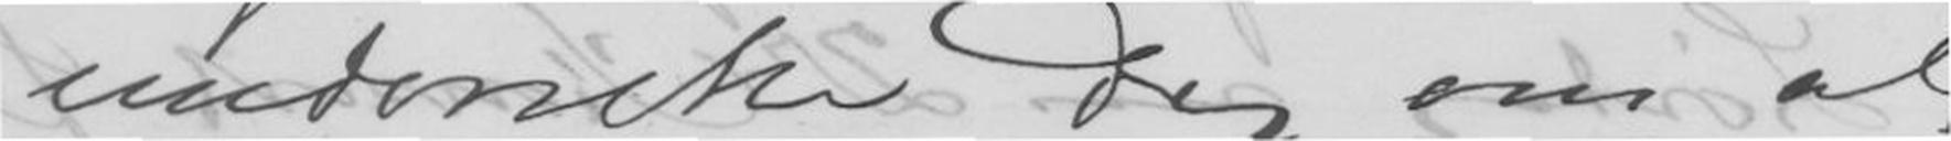underette Dig om at
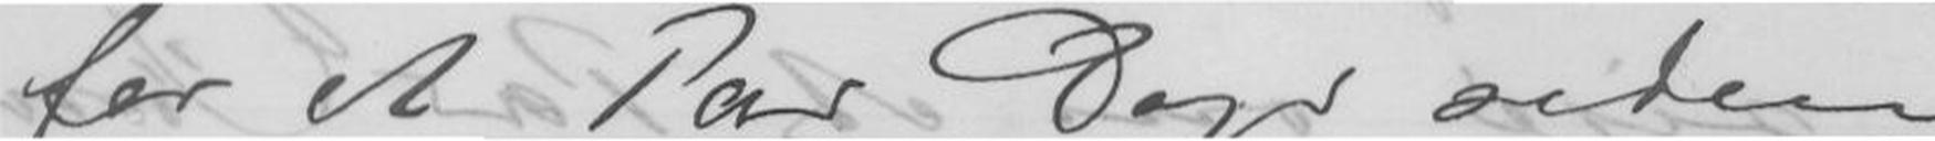for et Par Dage siden
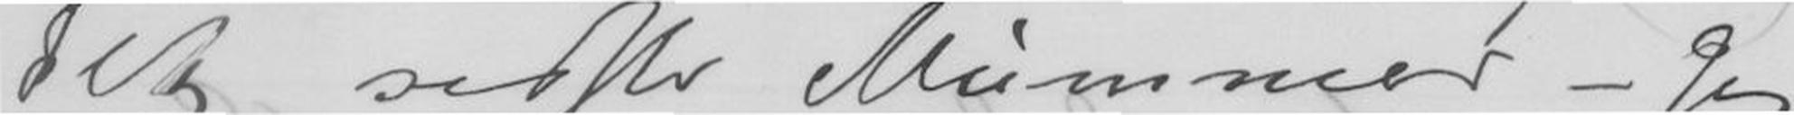det sidste Nummer - Jeg
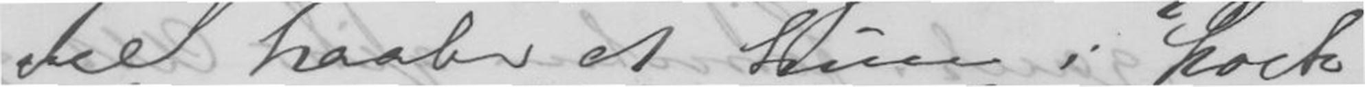vil haabe at hun i Stock-
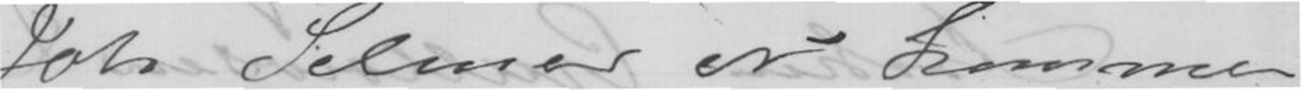Johs Selmer er kommen
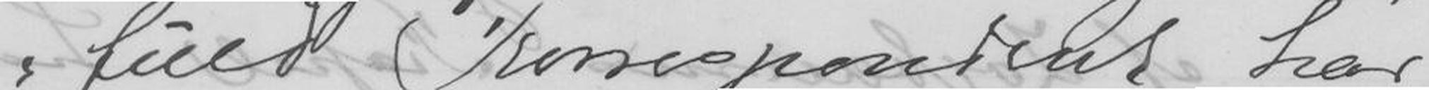fuld Korrespondent har
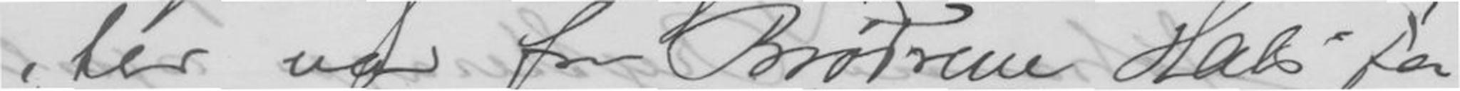ter var fra Brødrene Hals' Fa-
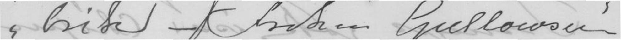brik - Frøken Gullowsen
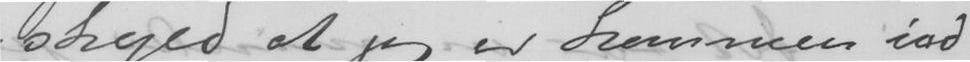skyld at jeg er kommen ind
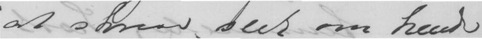at skrive slik om hende
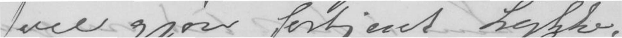vil gjøre fortjent Lykke,
